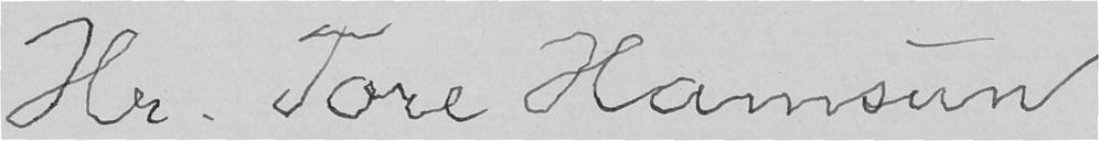Hr. Tore Hamsun
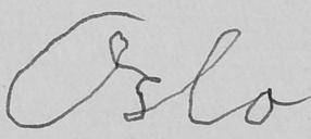Oslo
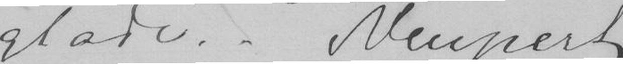glade. Neupert
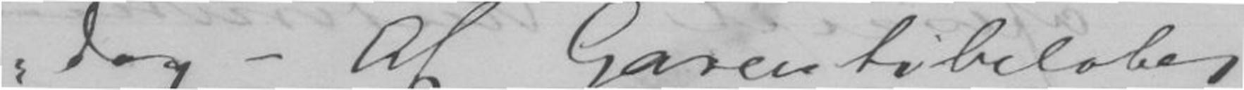dag - Af Garantibeløbet
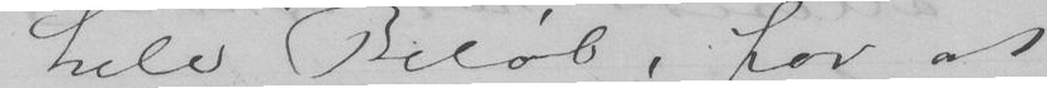hele Beløb, for at
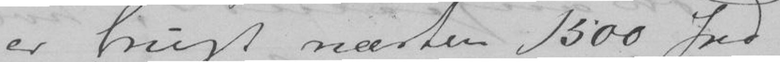er brugt næsten 1500 Spd
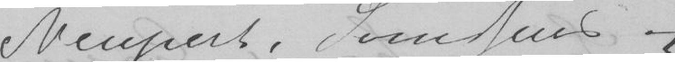Neupert, Svendsen og
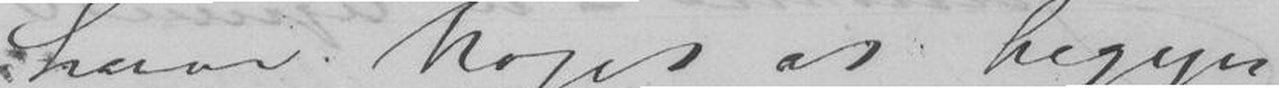have Noget at begyn
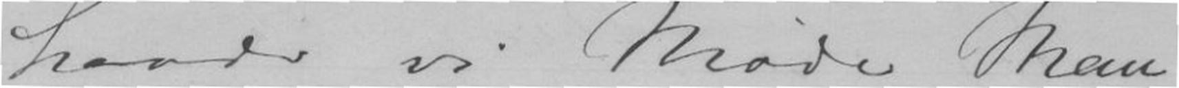havde vi Møde Man-
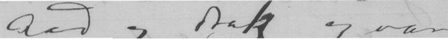Aad og drak og var
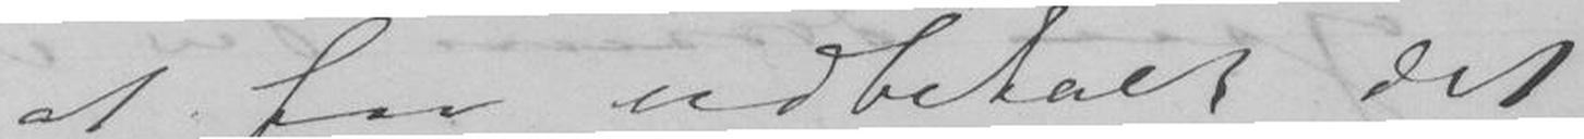at faa udbetalt det
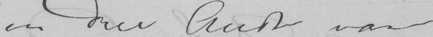en Del Andre var
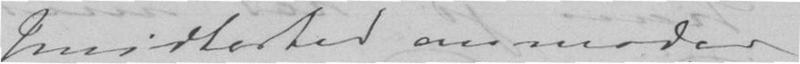Imidlertid anmoder
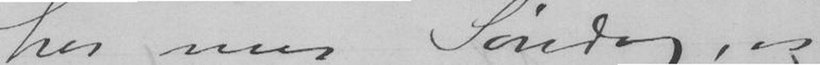hos mig Søndag, og
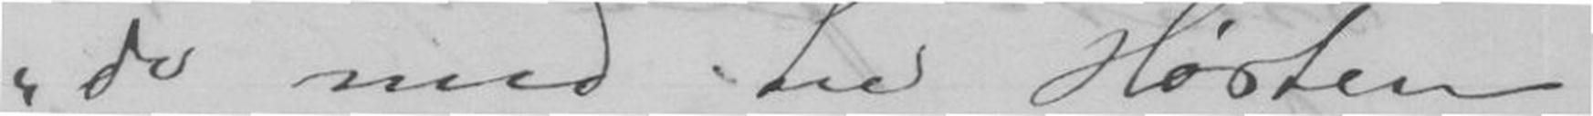de med til Høsten
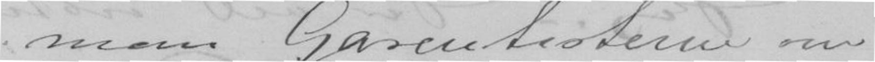man Garentisterne om
